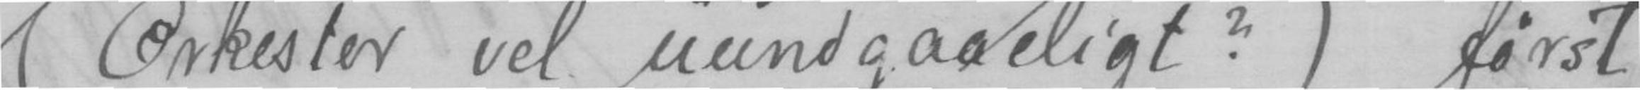(Orkester vel uundgaaeligt?) først
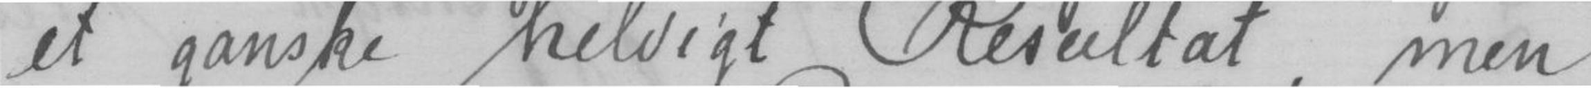et ganske heldigt Resultat, men
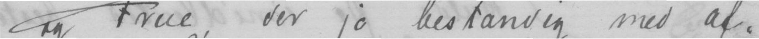og Fruen, der jo bestandig med af-
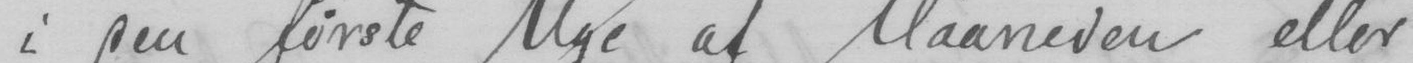i den første Uge af Maaneden eller
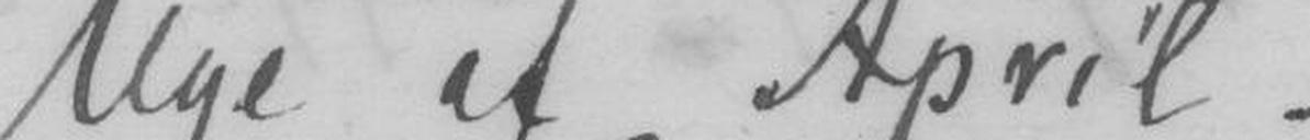Uge af April.
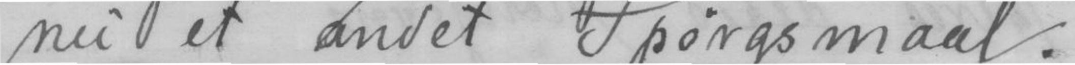nu et andet Spørsmaal.
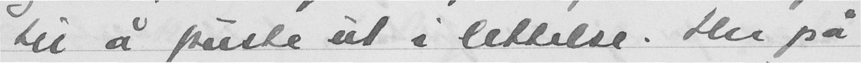til å puste ut i lettelse. Her på
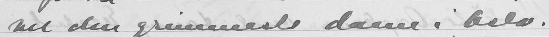nu den grimmeste dame i bilo.
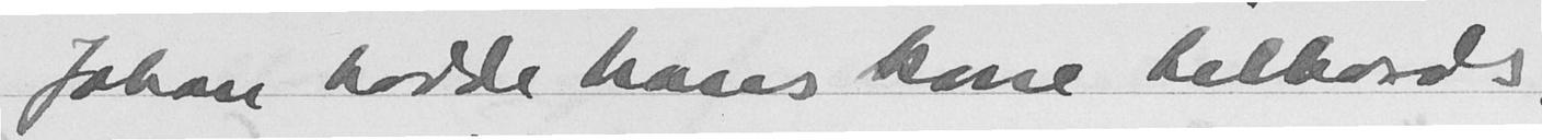Johan hadde hans kone tilbords
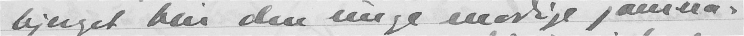bjerget blir den unge modige jenta-
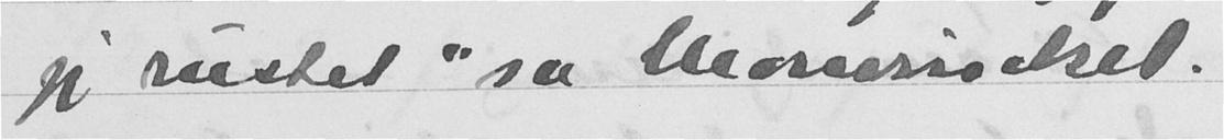jeg rustet" sa Mowinckel.
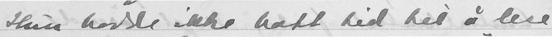Hun hadde ikke hatt tid til å lese
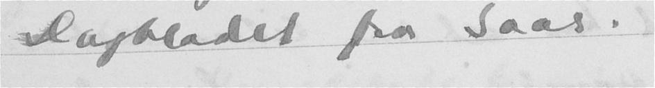Dagbladet fra Saas. -
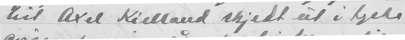til Axel Kielland skjeldt ut i tyske
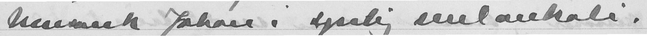havnet Johan i spydig melankoli.
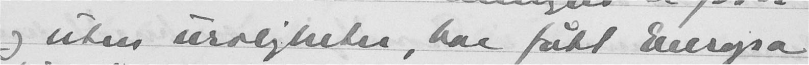og uten uroligheter, har fått Evesonja
